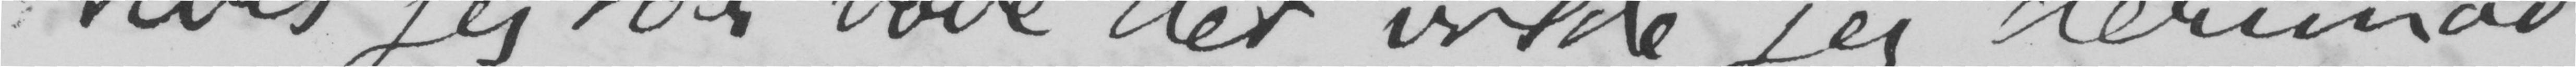Hvis jeg tør vove det, vilde jeg derimod
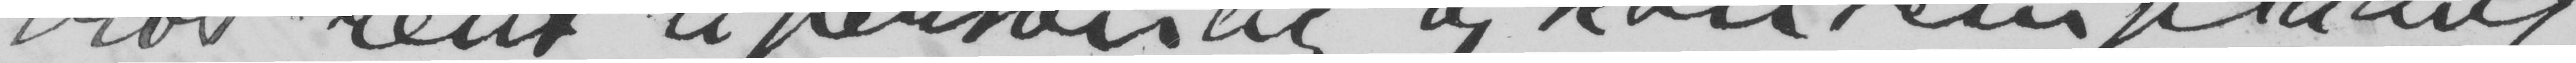blot rent upersonlig og kontemplativt,
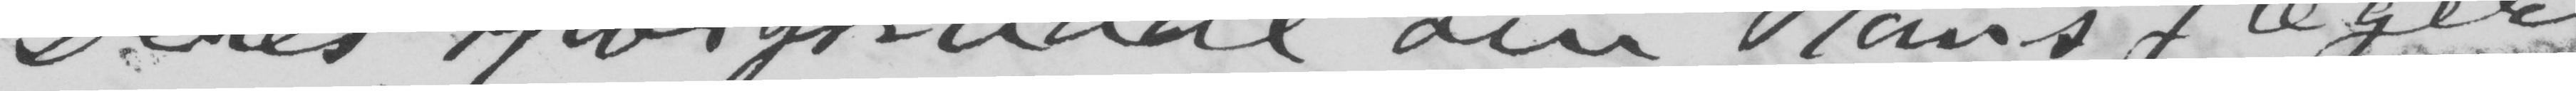Deres spørgsmaal om Hans Jæger,
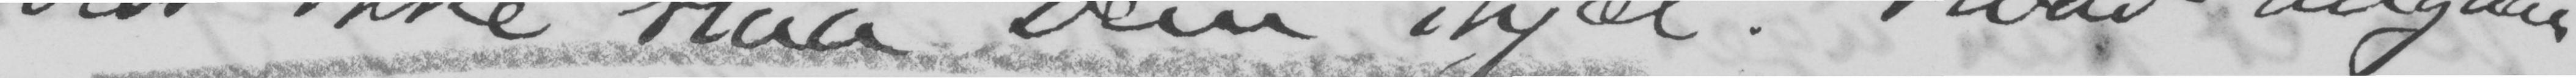blot ikke slaa Dem ihjæl! Hvad angaar
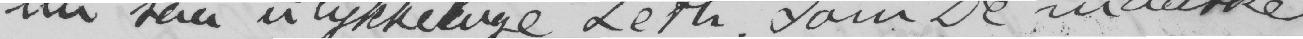nu saa ulykkelige Leth. Som De maaske
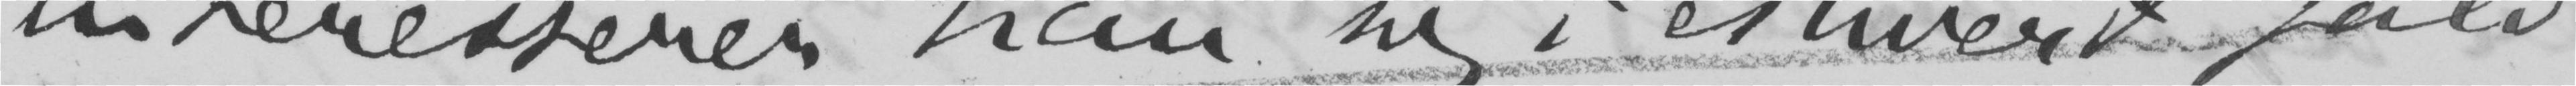interesserer han sig i ethvert fald
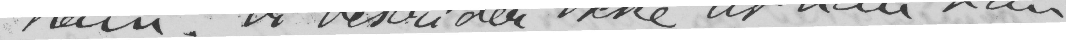ham. Vi bestrider ikke, at han kan
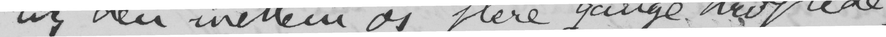lig den mellem os flere gange drøftede,
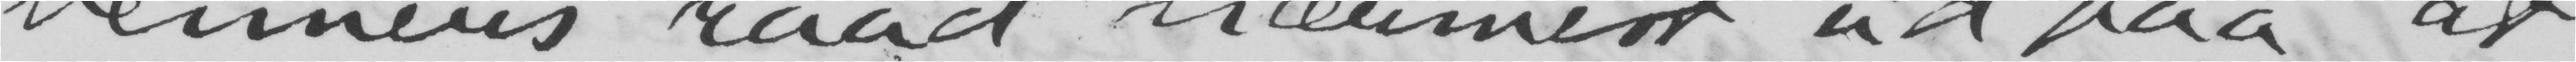venners raad nærmest ud paa, at
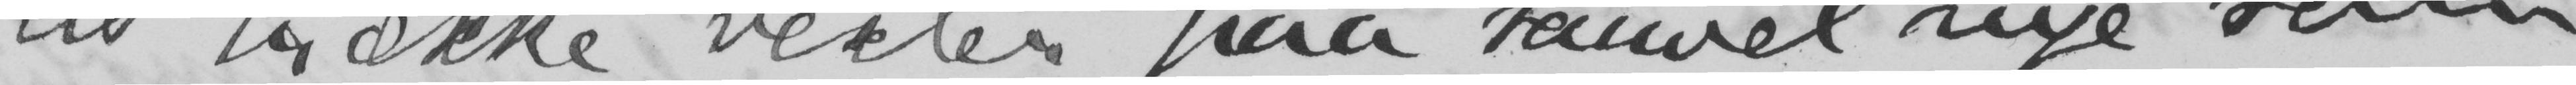at trække vexler paa saavel nye som
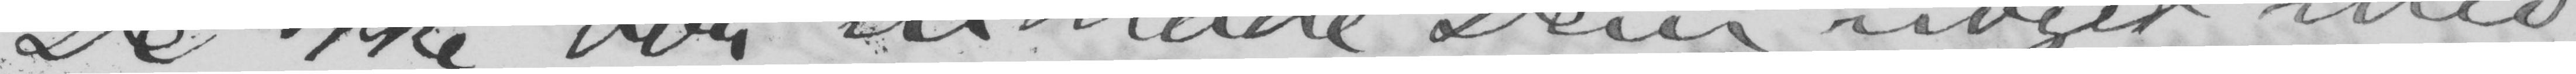De ikke bør indlade Dem noget med
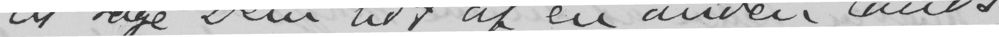at tage Dem lidt af en anden lands-
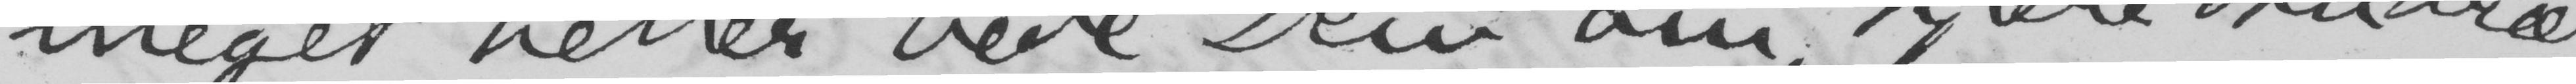meget heller bede Dem om, kjære Andræ,
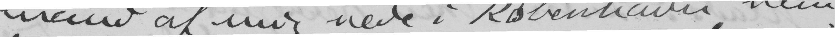mand af mig nede i København, nem-
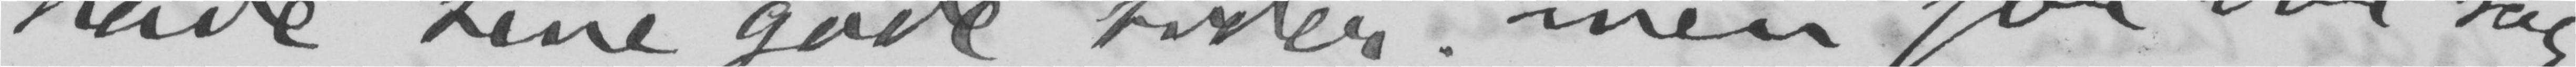have sine gode sider; men for vor sag
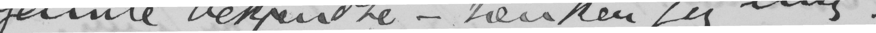gamle bekjendte – tænker jeg mig.
